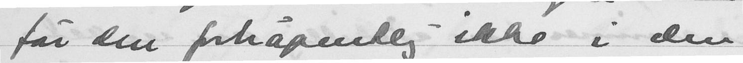får den forhåpentlig ikke i den
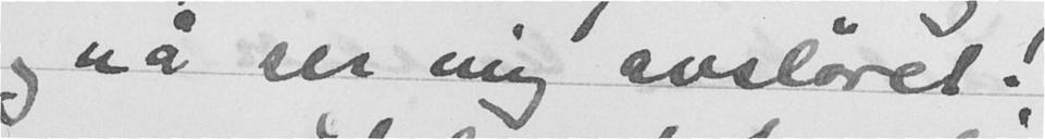og nå ser mig avsløret!
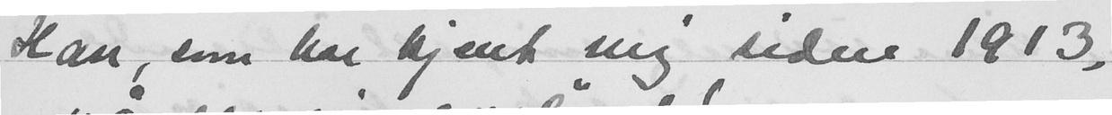Han, som har kjent mig siden 1913,
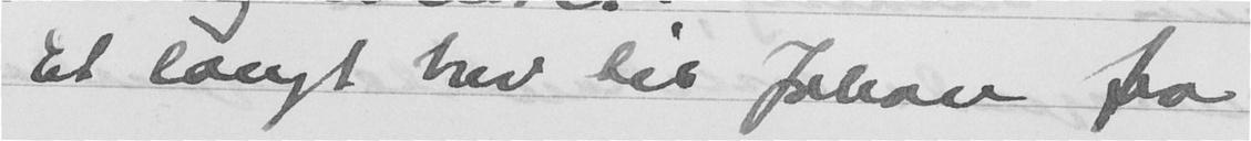Et langt brev til Johan fra
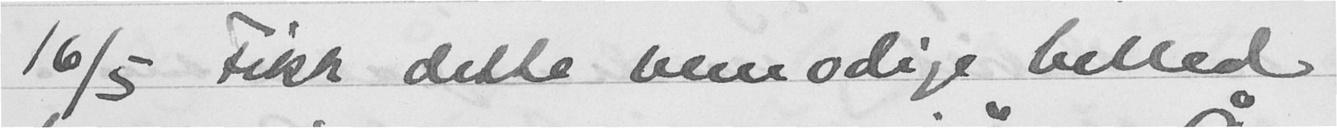16/5 Fikk dette vemodige billed
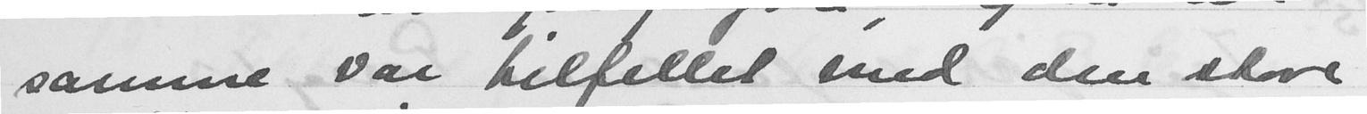samme var tilfellet med den store
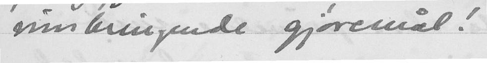innbringende gjøremål!
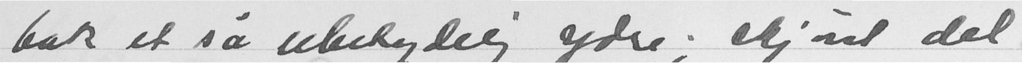bak et så ubetydelig ydre; skjønt det
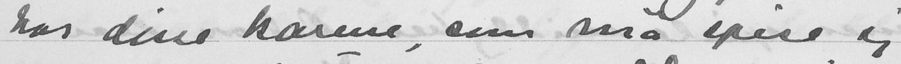hos disse karene, som må spise sig
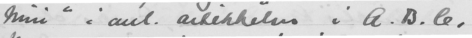Nini" i anl. artikkelen i A. B. C,
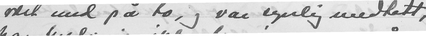vært med på to, og var synlig medtatt,
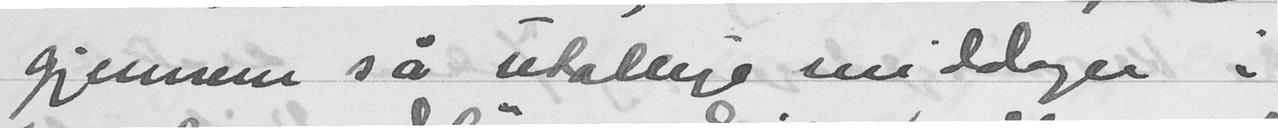gjennom så utallige middager i
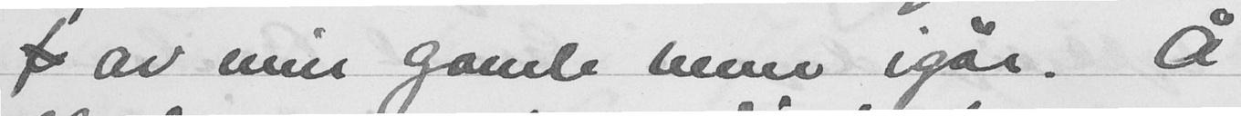av min gamle venn igår. Å,
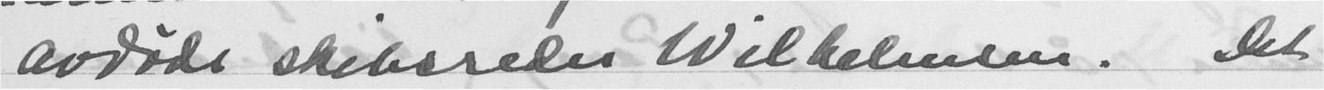avdøde skibsreder Wilhelmsen. Det
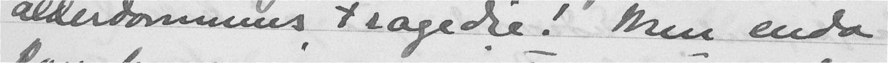alderdommens tragedie! Men enda
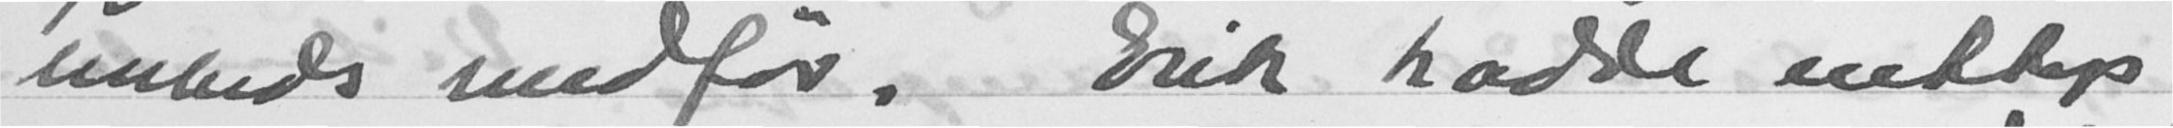mubeds medfør. Erik hadde nettop
